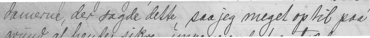damerne, der sagde dette, saa jeg meget op til paa
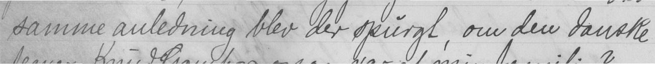samme anledning blev der spurgt, om den danske
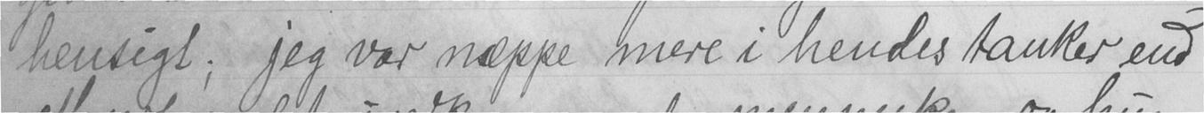hensigt; jeg var næppe mere i hendes tanker end
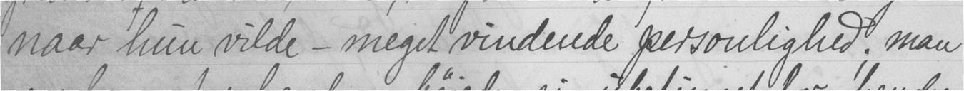naar hun vilde - meget vindende personlighed; man
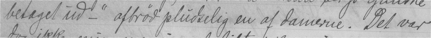betaget ud -" afbrød pludselig en af damerne. Det var
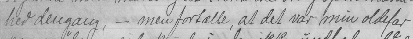hed dengang, - men fortælle, at det var min oldefar
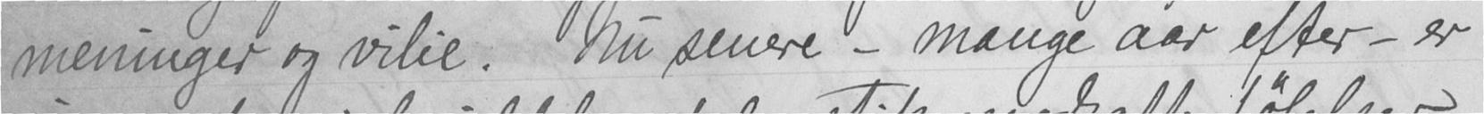meninger og vilie. Nu senere - mange aar efter - er
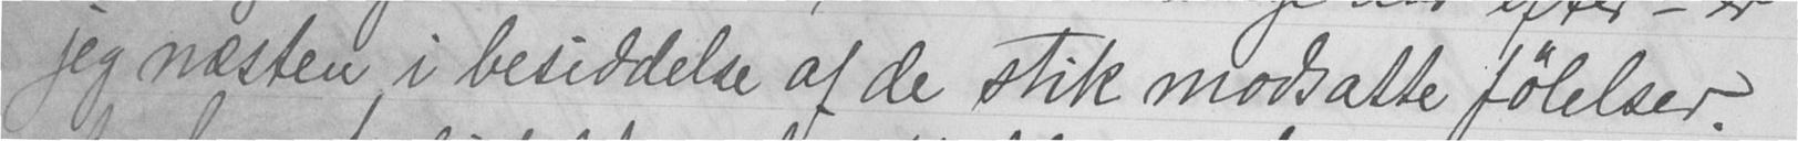jeg næsten i besiddelse af de stik modsatte følelser.
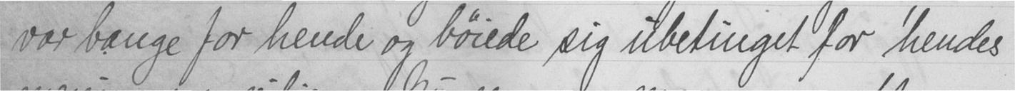var bange for hende og bøiede sig ubetinget for hendes
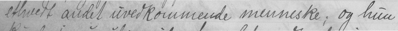ethvert andet uvedkommende menneske; og hun
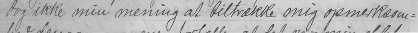dog ikke min mening at tiltrække mig opmerksom-
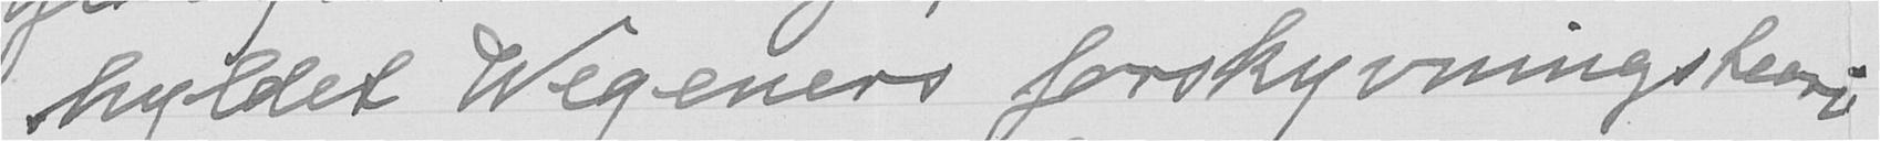hyldet Wegeners forskyvningsteori
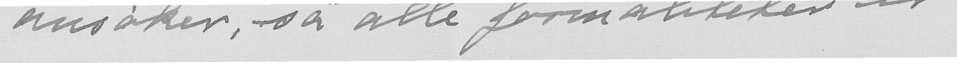ansøker så alle formaliteter er
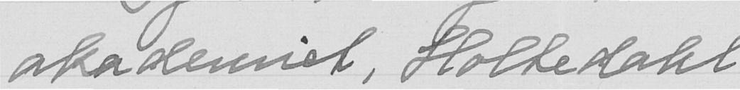akademiet, Holtedahl
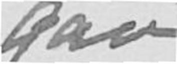gav
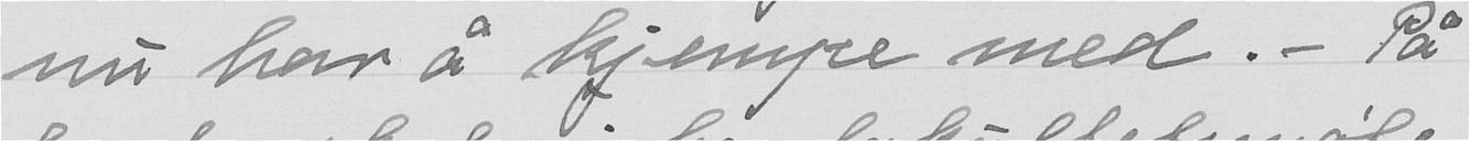nu har å hjempe med. - På
